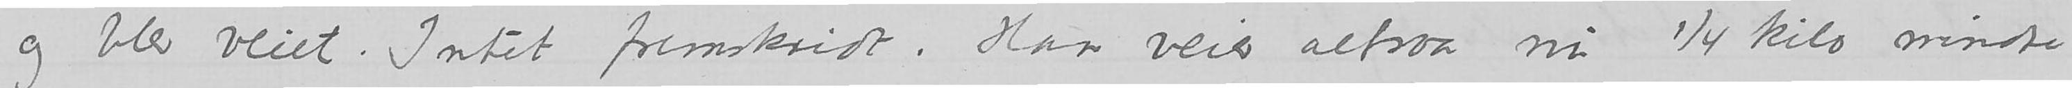og blev veiet. Intet fremskridt. Han veier altsaa nu ¼ kilo mindre
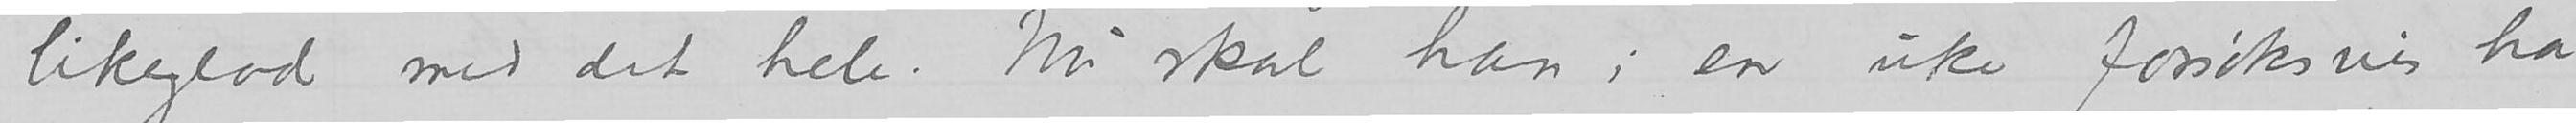likeglad med det hele. Nu skal han i en uke forsøksvis ha
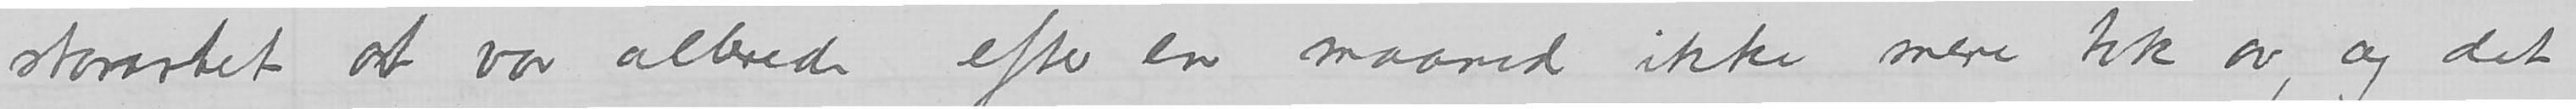storartet at vor allerede efter en maand ikke mere tok av, og det
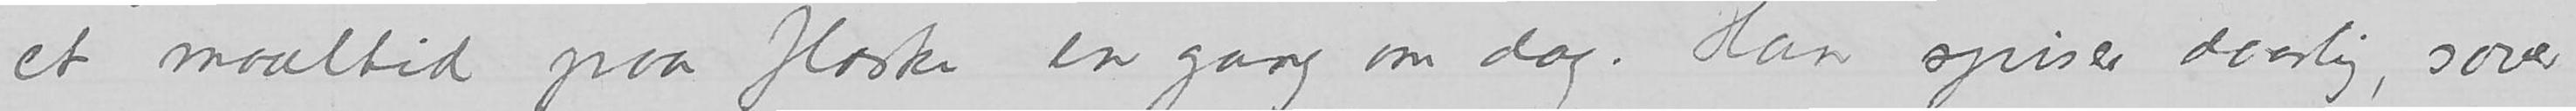et maaltid paa flaske en gang om dag. Han spiser daarlig, sover
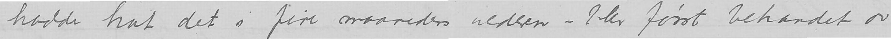hadde hat det i fire maaneders alderen – blev først behandlet av
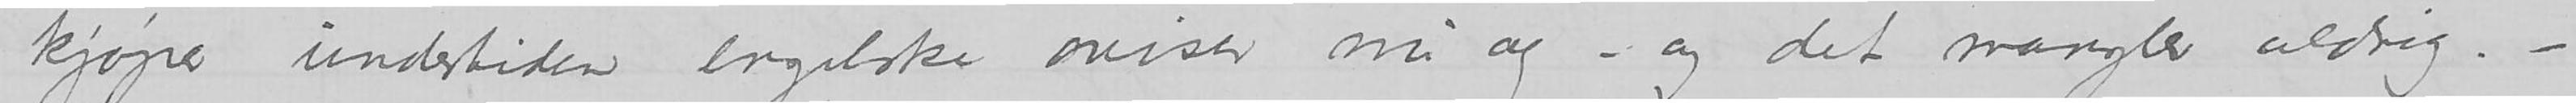kjøper undertiden engelske aviser nu og – og det mangler aldrig.  –
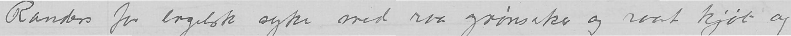Randers for engelsk syke med raa grønsaker og raat kjøt og
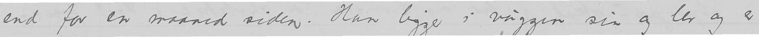end for en maaned siden. Han ligger i vuggen sin og ler og er
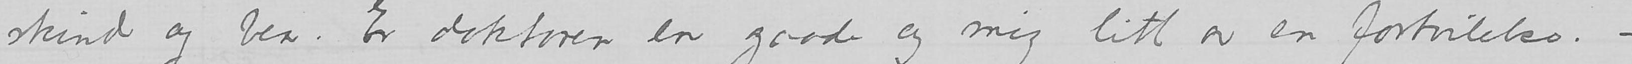skind og ben. Er doktoren en gaade og mig litt av en fortvilelse. –
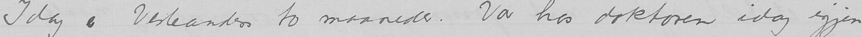Idag er Vesleanders to maaneder. Var hos doktoren idag igjen
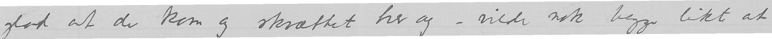glad at de kom og skvættet her og – vilde nok begge likt at
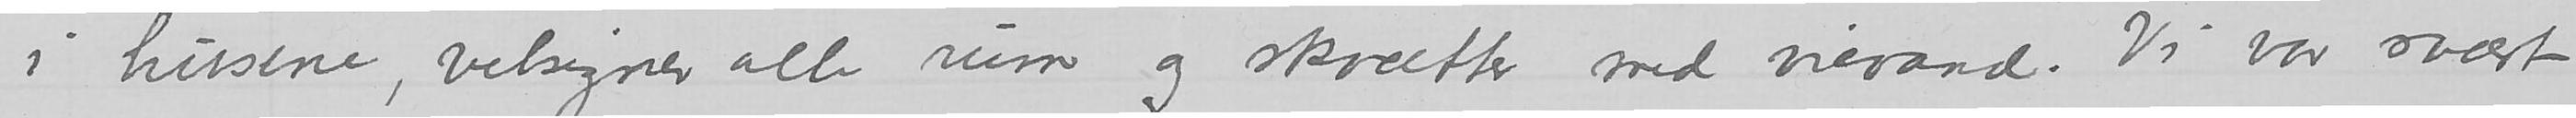i husene, velsigner alle rum og skvætter med vievand. Vi var svært
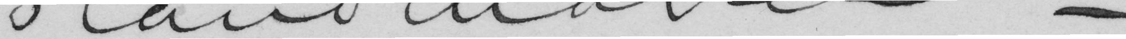Scandinavien –
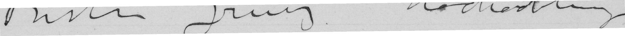Bester Grusz Hochachtung
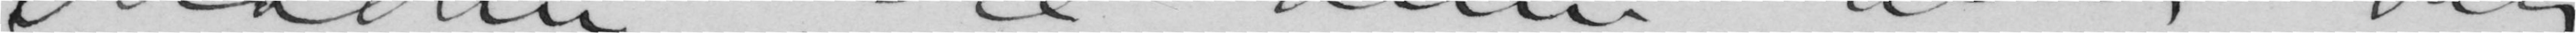Machen Sie denn alles dasz
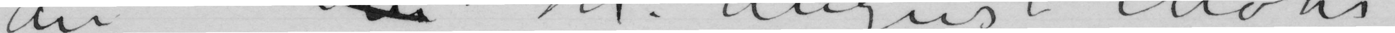an Wen Hr. August Mohr
